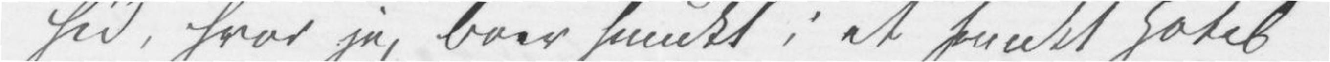hid, hvor jeg boer smukt i et smukt Hotel
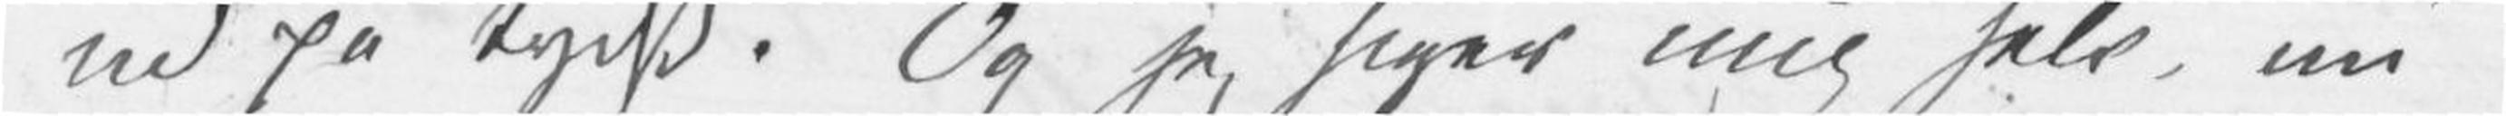ud på tydsk. Og jeg siger mig selv, nu
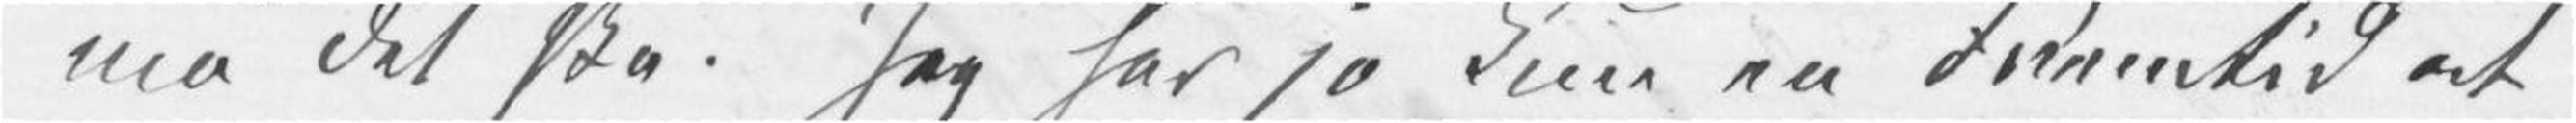må det ske. Jeg har jo kun en Fremtid at
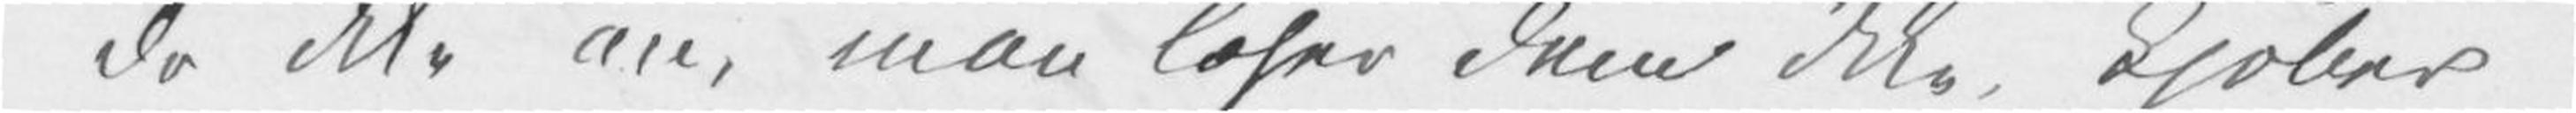de ikke an, man læser dem ikke, kjøber
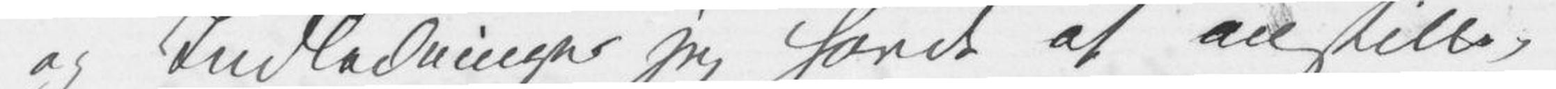og Indledninger jeg havde at anstille,
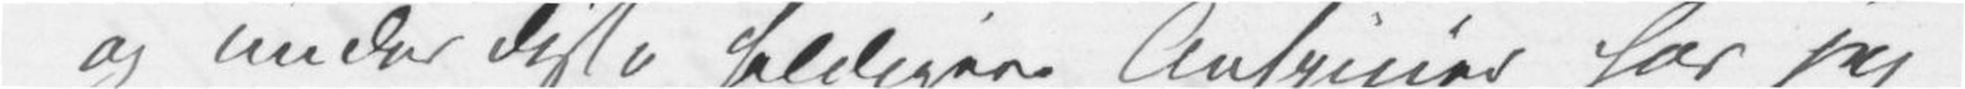og under disse heldigere Auspicier har jeg
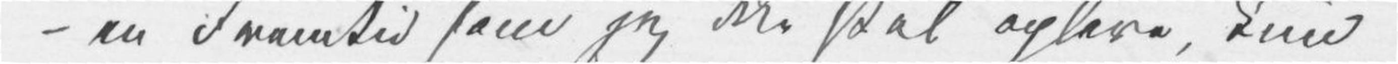– en Fremtid som jeg ikke skal opleve, kun
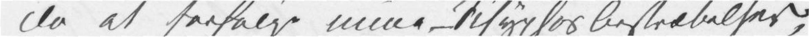da at forfølge mine Sisyphosbestræbelser,
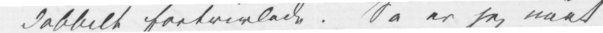dobbelt fortvivlede. Så er jeg nået
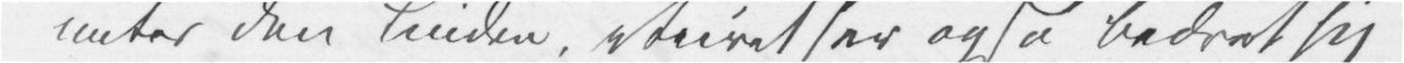«unter den Linden». Veiret har også bedret sig
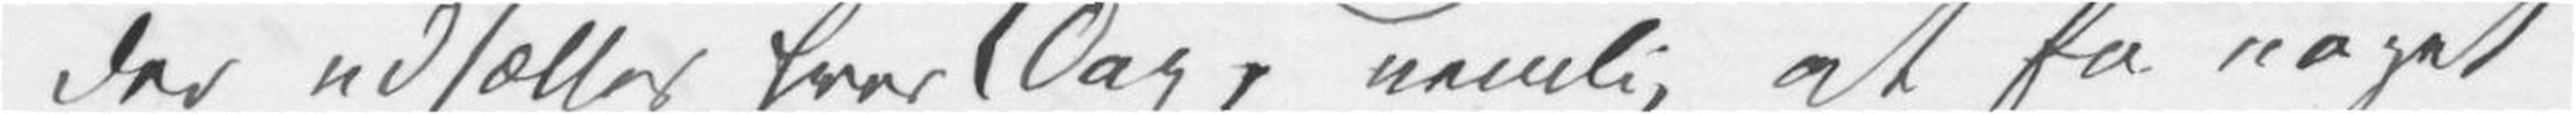der udsættes hver Dag, nemlig at få noget
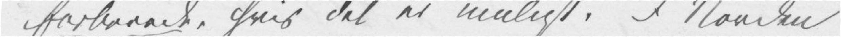forberede, hvis det er muligt. I Norden
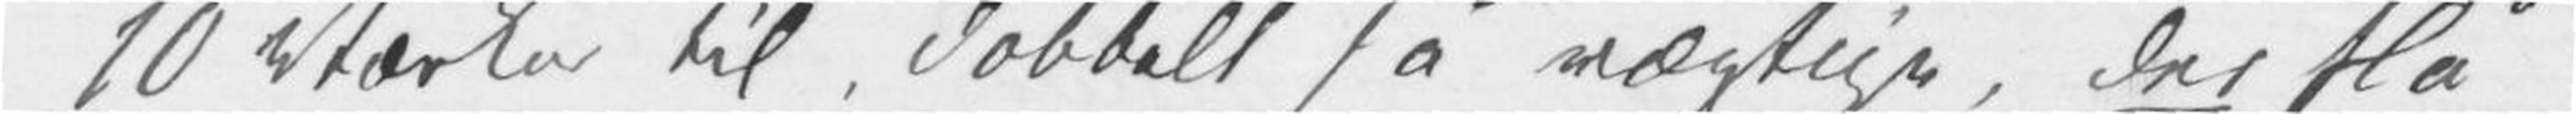10 Værker til, dobbelt så vægtige, der slå
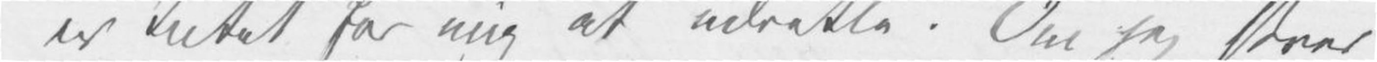er Intet for mig at udrette. Om jeg skrev
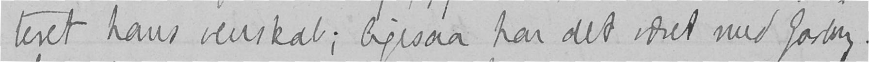teret hans venskab; ligesaa har det været med Garborg.
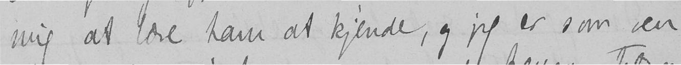mig at lære ham at kjende, og jeg er som ven
